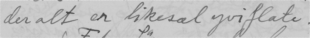der alt er likesæl yviflate
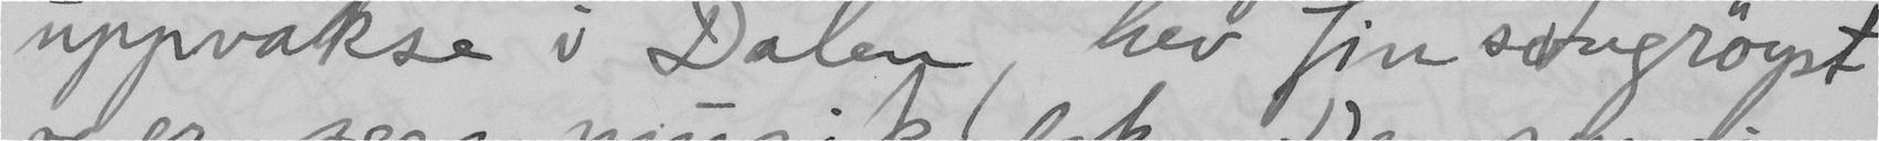uppvakse i Dalen hev fin songröøyst
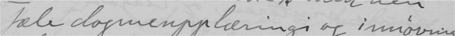fæle dogmeupplæringi og innöving
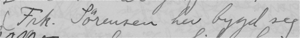(Frk. Sörensen hev bygd seg
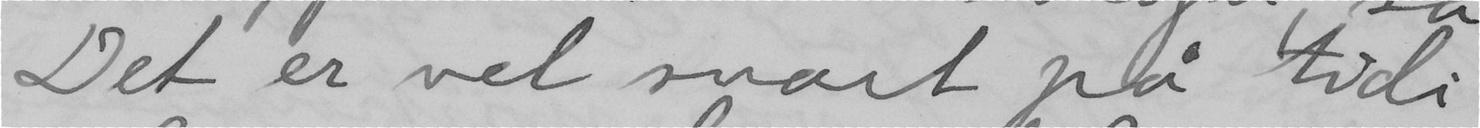Det er vel snart på tidi
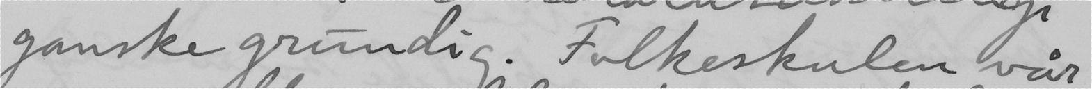ganske grundig. Folkeskulen vår
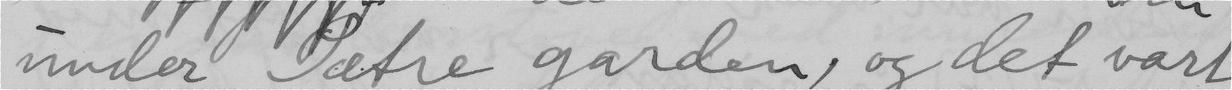under Sætre garden, og det vart
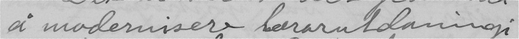å modernisere lerarutdanningi
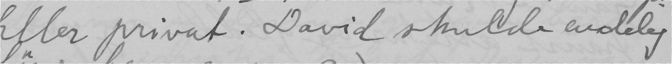eller privat. David skulde endeleg
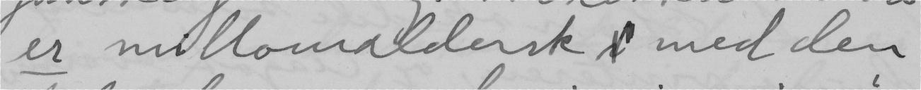er millomaldersk  med den
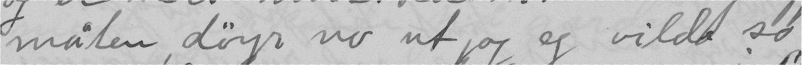måten döyr no ut, og eg vilda so
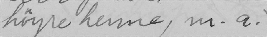höyre henne, m.a.)
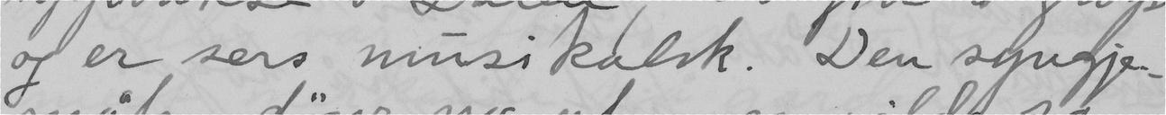og er sers musikalsk. Den syngje-
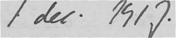7 dec. 1917.
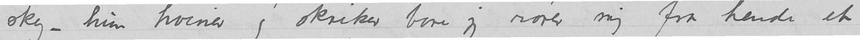sky – hun hviner og skriker bare jeg rører mig fra hende et
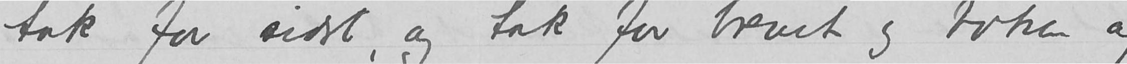Tak for sidst, og tak for brevet og boken og
